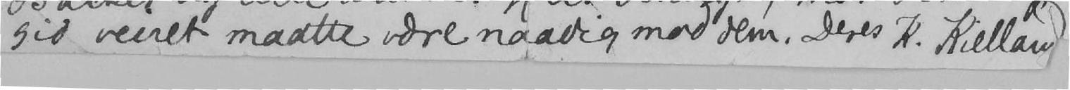gid veiret maatte være naadig mod dem. Deres K. Kielland
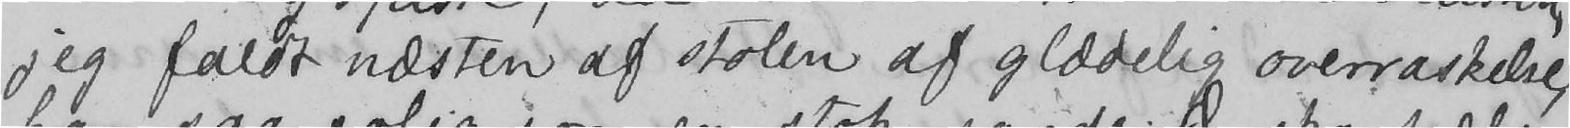jeg faldt næsten af stolen af glædelig overraskelse,
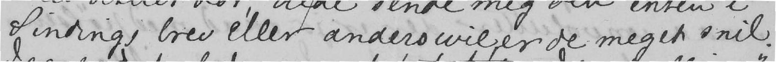Sindings brev eller anderswie, er de meget snil.
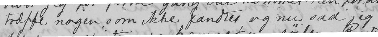træffe nogen, som ikke fandtes og nu sad jeg
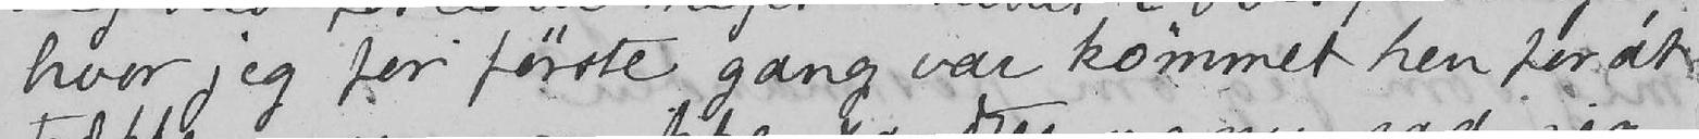hvor jeg for første gang var kommet hen for at
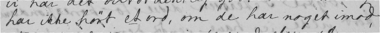har ikke hørt et ord, om de har noget imod,
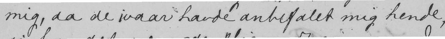mig, da de ivaar havde anbefalet mig hende,
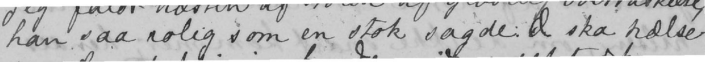han saa rolig som en stok sagde: Æ ska hælse
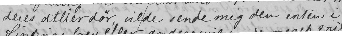deres atlierdør, vil de sende mig den enten i
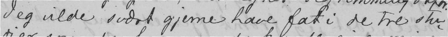Jeg vilde svært gjerne have fat i de tre stu-
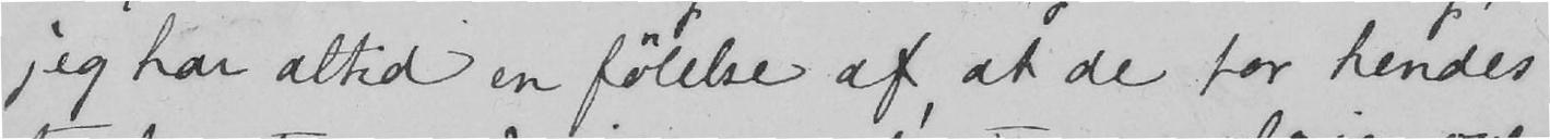jeg har altid en følelse af, at de for hendes
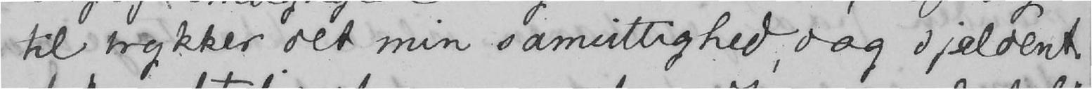til trykker det min samvittighed, dog sjeldent.
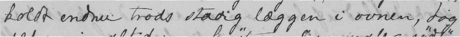koldt endnu trods stadig læggen i ovnen, dog
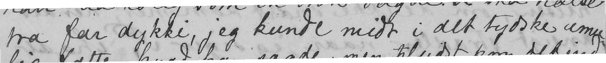fra far dykki, jeg kunde midt i det tydske umu-
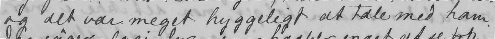og det var meget hyggeligt at tale med ham.
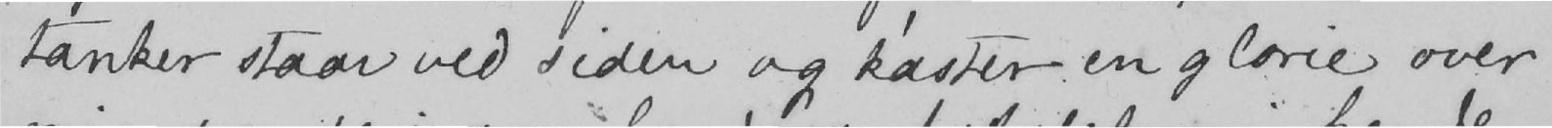tanker staar ved siden og kaster en glorie over
